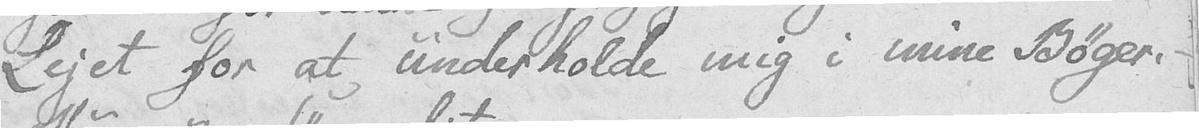Lejet for at underholde mig i mine Bøger. -
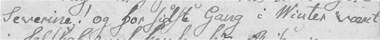Severine! og for sidste Gang i Vinter været
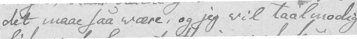det maae saa være, og jeg vil taalmodig
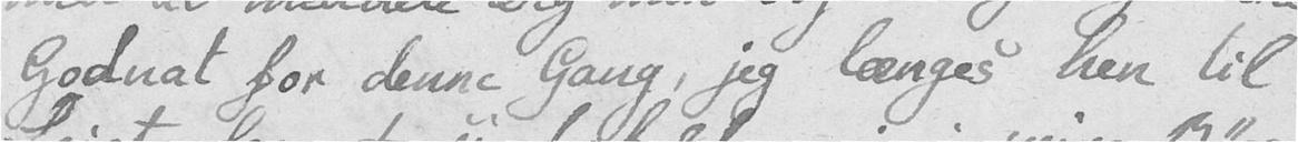Godnat for denne Gang, jeg længes hen til
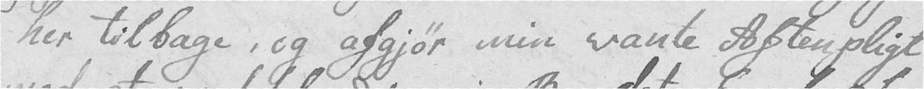her tilbage, og afgjør min vante Aftenpligt
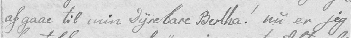afgaae til min Dyrebare Bertha! nu er jeg
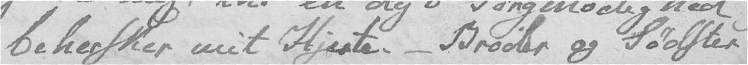behersker mit Hjerte. – Broder og Sødster
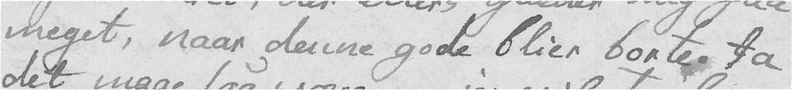meget, naar denne gode blier borte. Ja
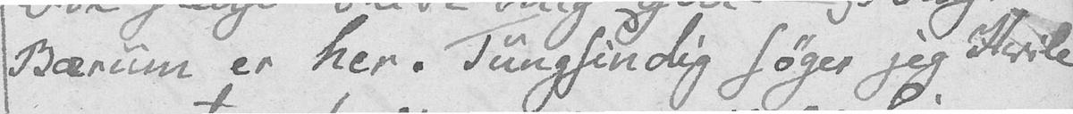Bærum er her. Tungsindig søger jeg Hvile
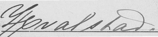Hvalstad
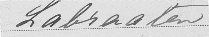Labraaten
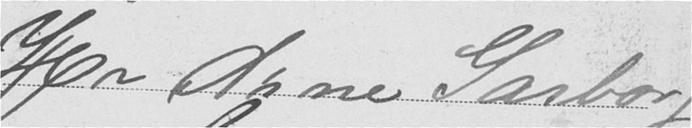Hr Arne Garbor
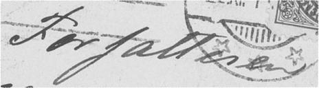Forfatteren
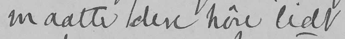maatte dere høre lidt
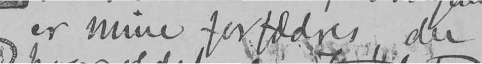er mine forfædres, der
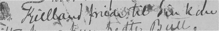Kielland friede til sin kone
